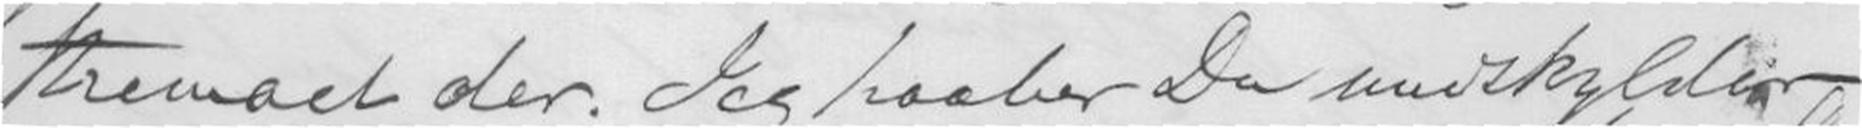themaet der. Jeg haaber Du undskylder
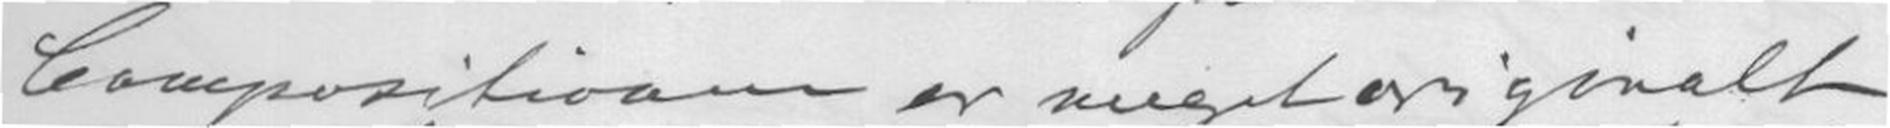Compositionen er meget originalt
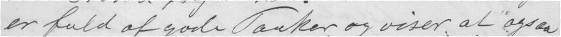er fuld af gode Tanker og viser, at ogsaa
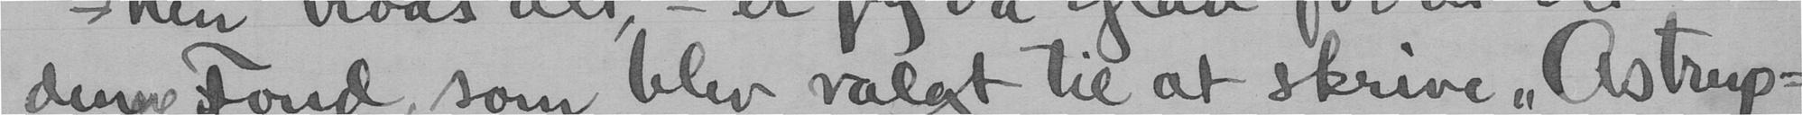denne Fond, som blev valgt til at skrive "Astrup-
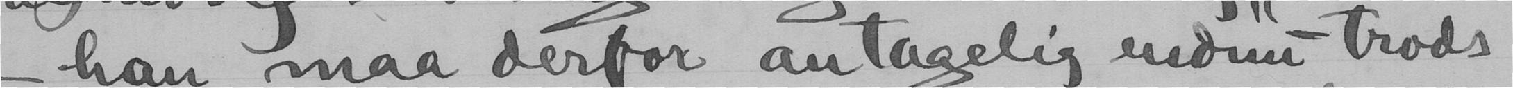- han maa derfor antagelig endnu - trods
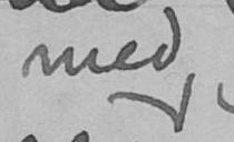med
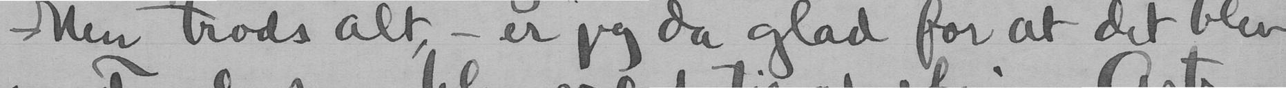Men trods alt - er jeg da glad for at det blev
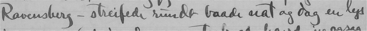Ravensberg - streifede rundt baade nat og dag en lys
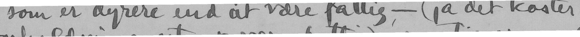som er dyrere end at være fattig - (ja det koster
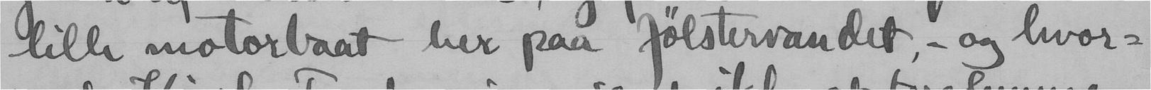lille motorbaat her paa Jølstervandet - og hvor-
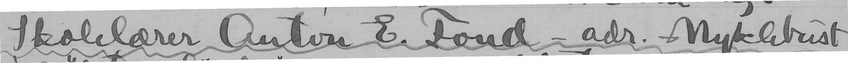Skølelærer Anton E. Fond - adr. Myklebust
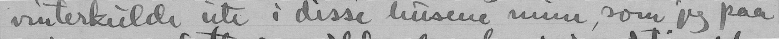vinterkulde ute i disse husene mine, som jeg paa
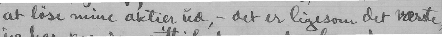at løse mine aktier ud - det er ligesom det værste
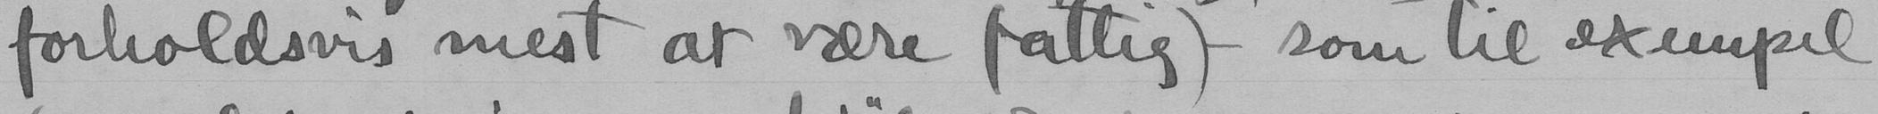forholdsvis mest at være fattig) som til exempel
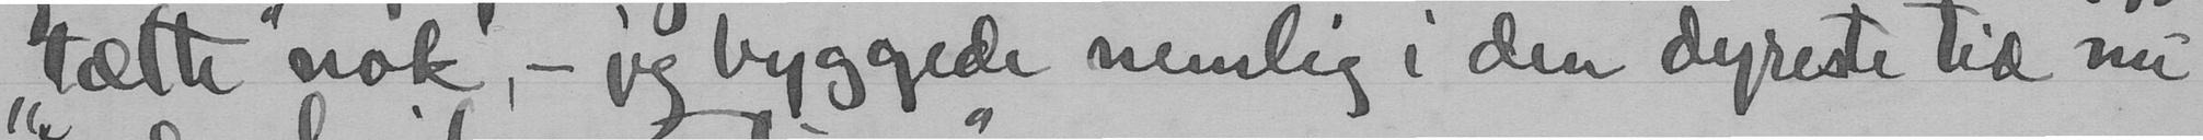"tætte" nok, - jeg byggede nemlig i den dyreste tid nu
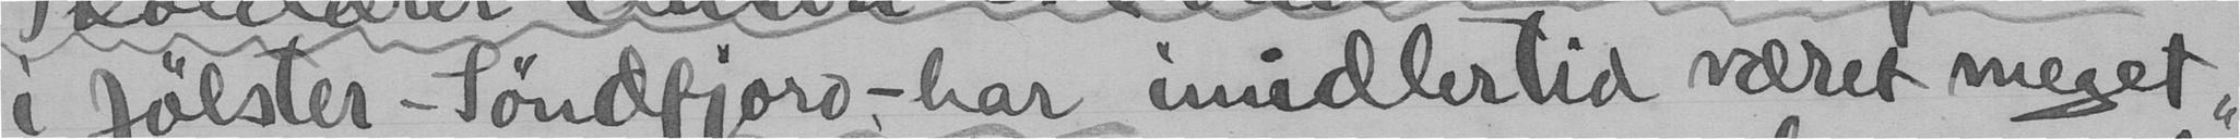i Jølster - Søndfjord, - har imidlertid været meget
